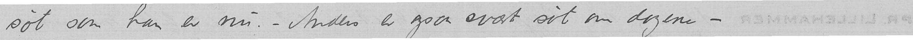søt som han er nu. – Anders er ogsaa svært søt om dagen –
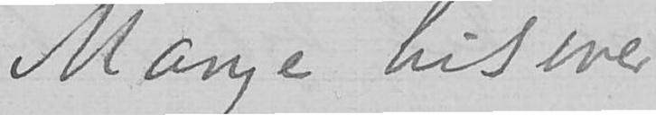Mange hilsener
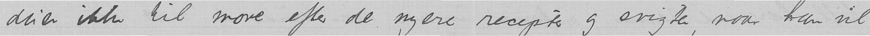duer ikke til move efter de nyere recepter og svigter, naar han vil
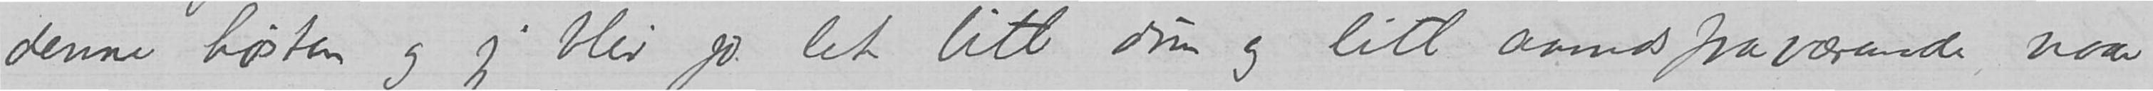denne høsten og jeg blir jo let litt dum og litt aandsfraværende naar
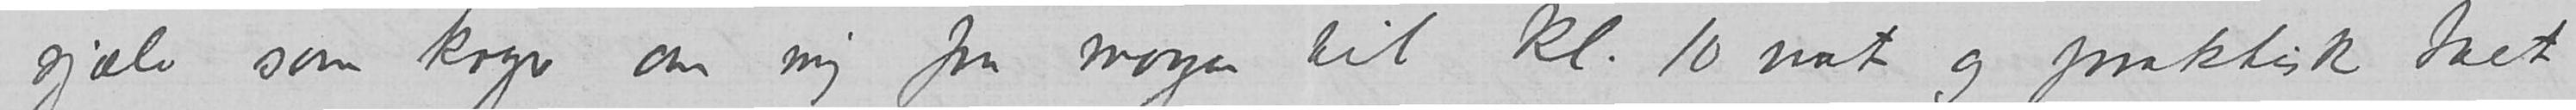sjæler som kryr om mig fra morgen til kl. 10 nat og praktisk talt
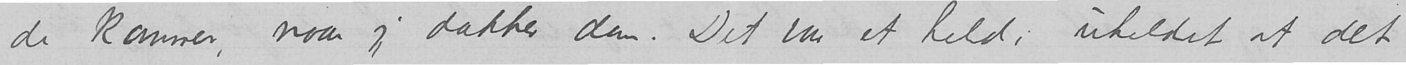de kommer, naar jeg dækker dem. Det var et held i uheldet at det
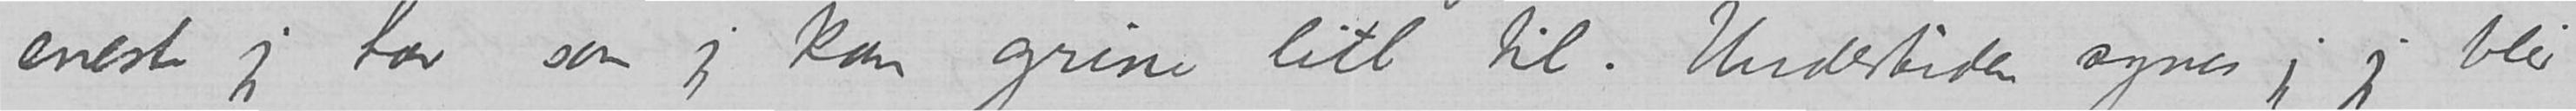eneste jeg har som jeg kan grine litt til. Undertiden synes jeg jeg blir
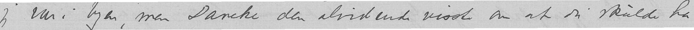jeg var i byen, men Dancke den alvidende visste om at du skulde ha
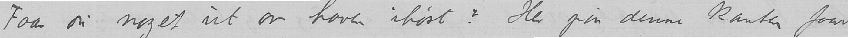Faar du noget ut av haven ihøst? Her paa denne kanten faar
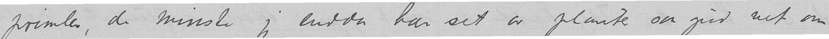primler, de minste jeg endda har set av planter saa gud vet om
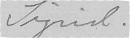Sigrid.
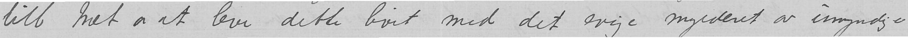litt træt av at leve dette livet med det evige mylderet av umyndige
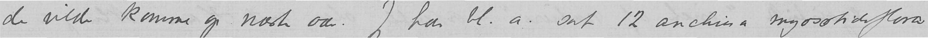du vilde komme op næste aar. Jeg har bl.a. sat 12 anchina myosætidsflora
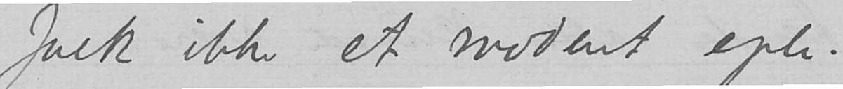folk ikke et modent eple.
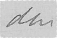din
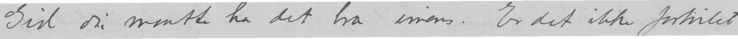Gid du maatte ha det bra imens. Er det ikke fortvilet
